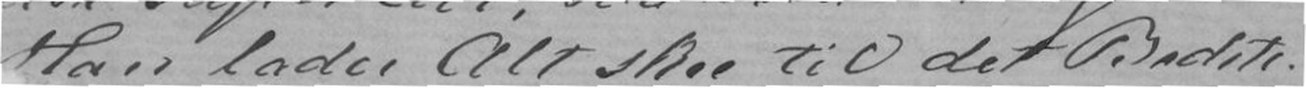Han lader Alt skee til det Bedste.
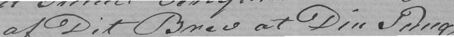af Dit Brev at Din Punget
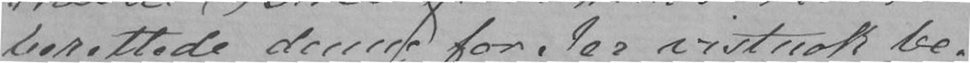berettede denne for Jer vistnok be-
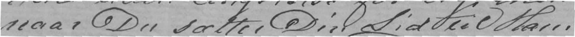naar Du sætter Din Lid til Ham
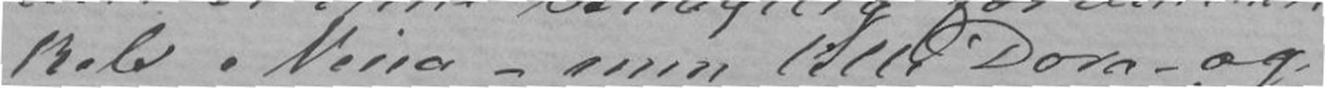kels Nina - min lille Dora - og
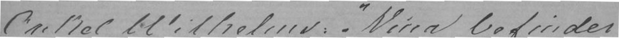Onkel Wilhelms: "Nina befinder
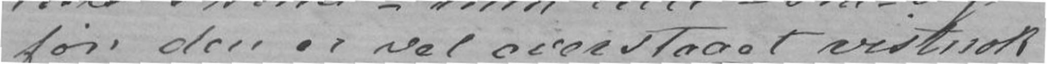før den er vel overstaaet vistnok
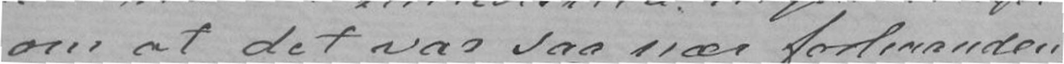om at det var saa nær forhaanden,
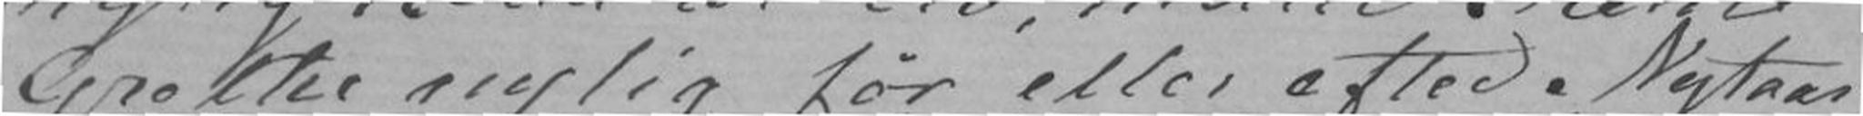Grethe nylig før eller efter Nytaar
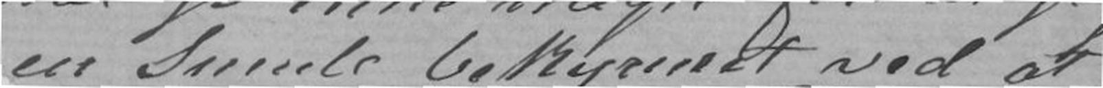en Smule bekymret ved at
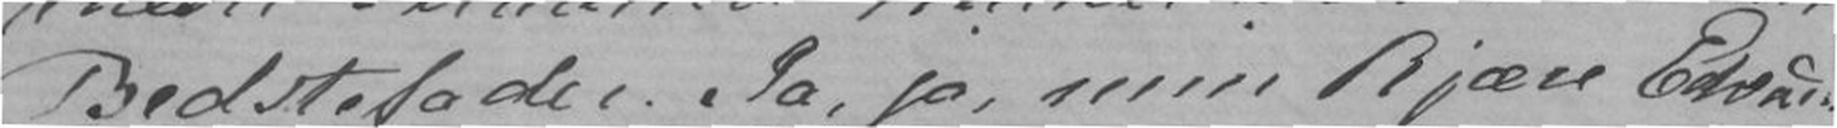Bestefader. Ja, ja, min kjære Edvard!
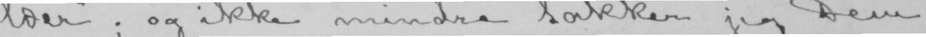læer»; og ikke mindre takker jeg Dem
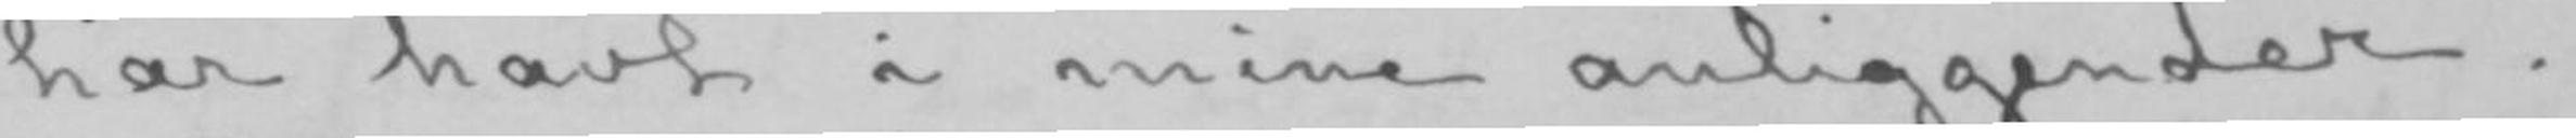har havt i mine anliggender.
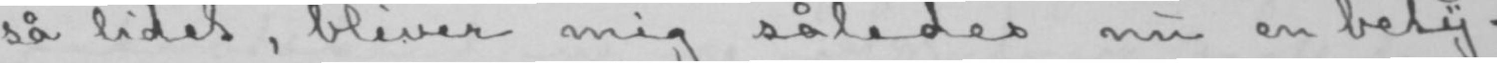så lidet, bliver mig således nu en bety-
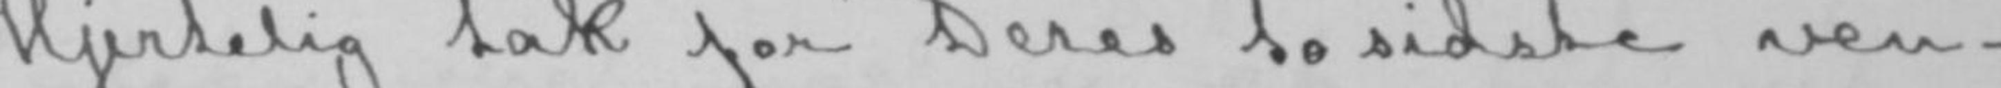Hjertelig tak for Deres to sidste ven-
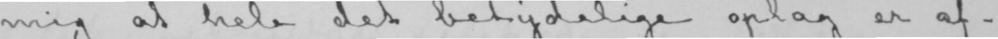mig at hele det betydelige oplag er af-
